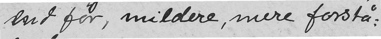end for, mildere, mere forstå-
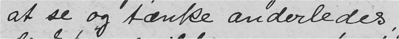at se og tænke anderledes,
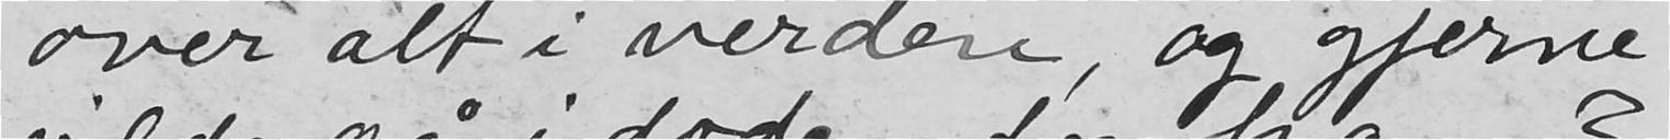over alt i verden, og gjerne
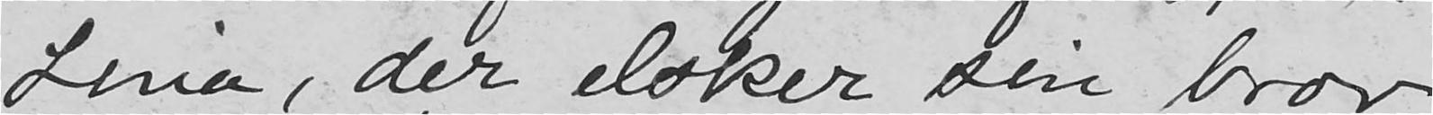Lina, der elsker sin bror
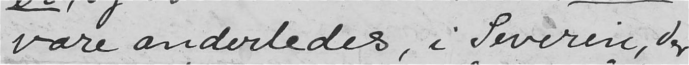være anderledes, i Severin, der
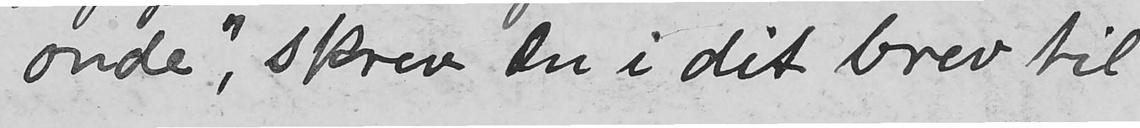onde, skrev Du i dit brev til
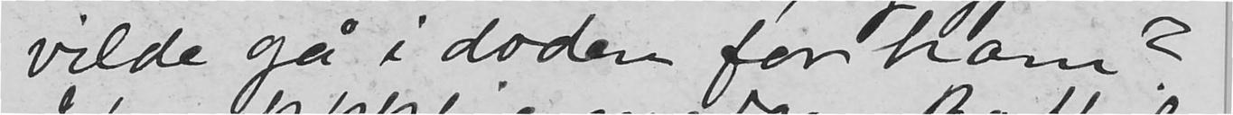vilde gå i døden for ham?
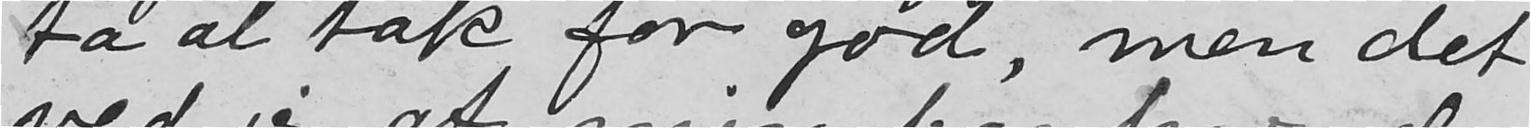ta at tak for god, men det
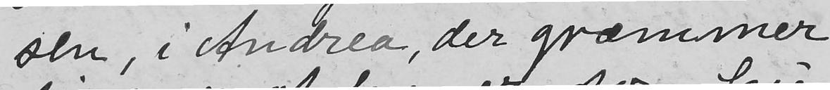sen, i Andrea, der græmmer
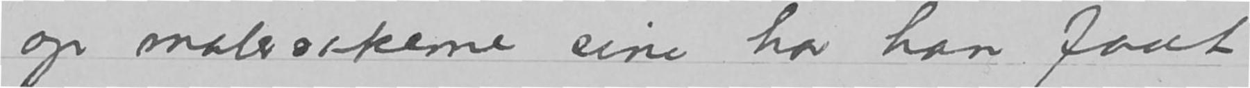op malersakerne sine har han faat
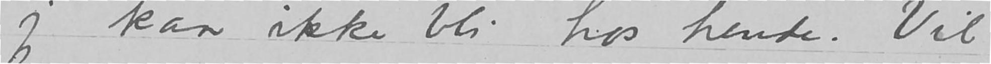jeg kan ikke bli hos hende. Vil
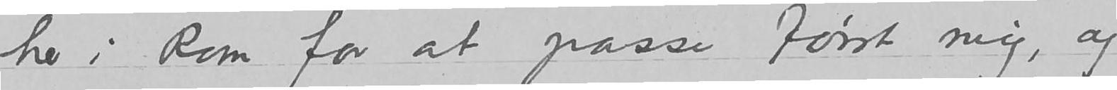her i Rom for at passe først mig, og
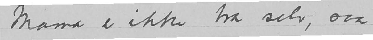Mama er ikke bra selv, saa
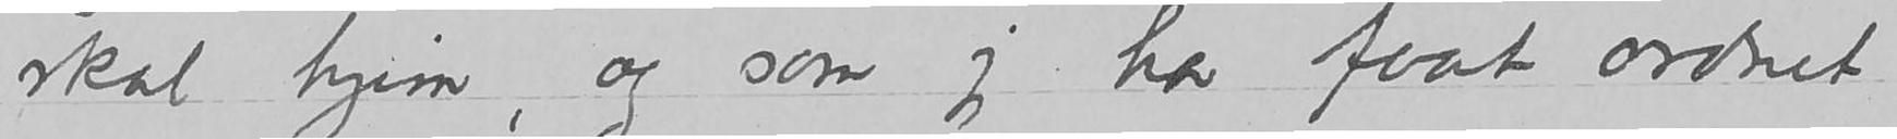skal hjem, og som jeg har faat ordnet
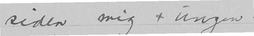siden mig + ungen.
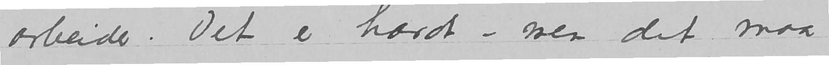arbeider. Det er hardt – men det maa
